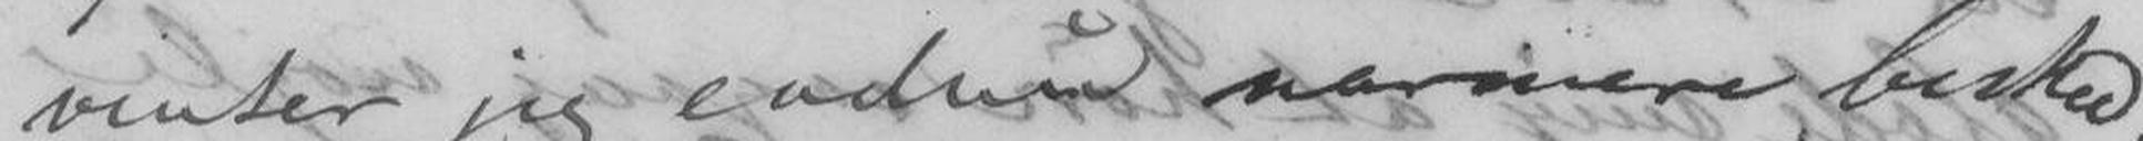venter jeg endnu nærmere besked,
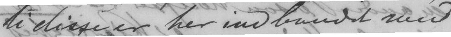ti disse er her indbundet med
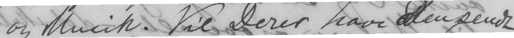og Musik. Vil Derer have Den sendt
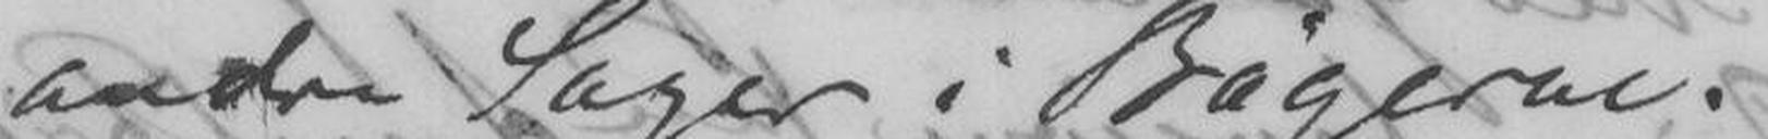andre Sager i Bøgerne.
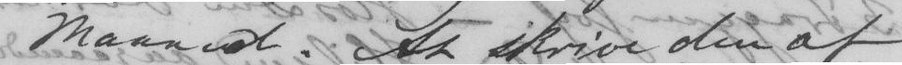Maaned. At skrive den af
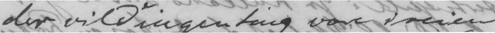der vilde ingen ting værer i veien
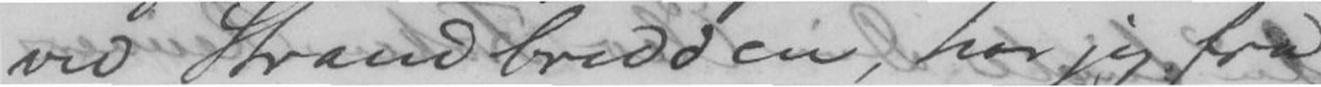ved Strandbredden; har jeg fra
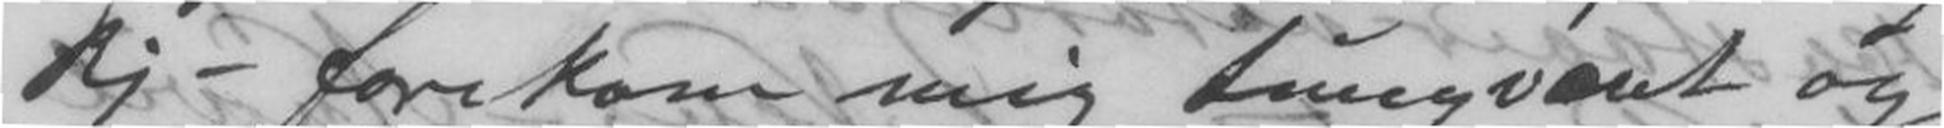dig - forekom mig tungvint og
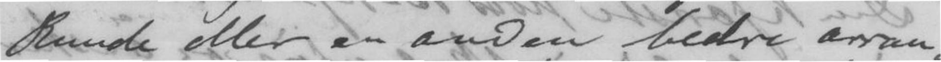kunde eller en anden bedre arran-
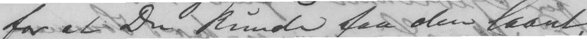for at Du kunde faa den laant,
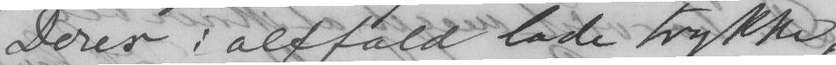Dereri altfald lade trykke,
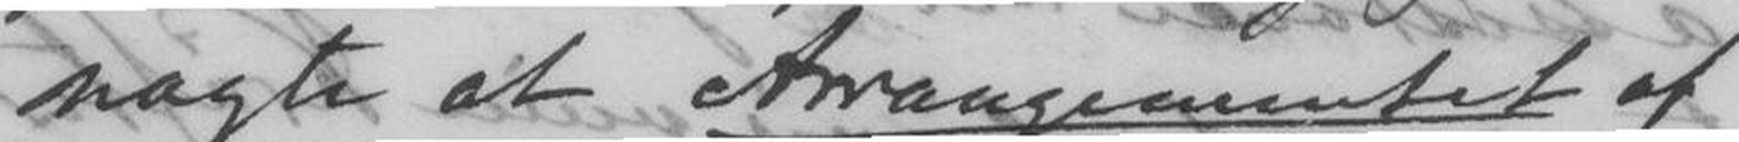nægte at Arrangementet af
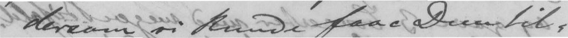dersom vi kunde faae Dem til-
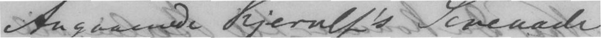Andgaaende Kjerulf's Serenade
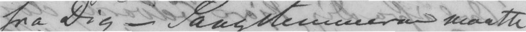fra Dig - Sanstemmen maatte
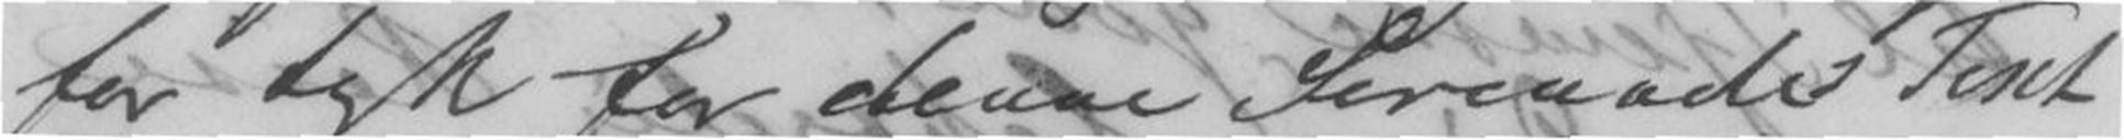for tyk for denne Serenad Text
